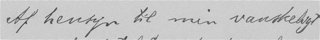Af hensyn til min vanskeligt
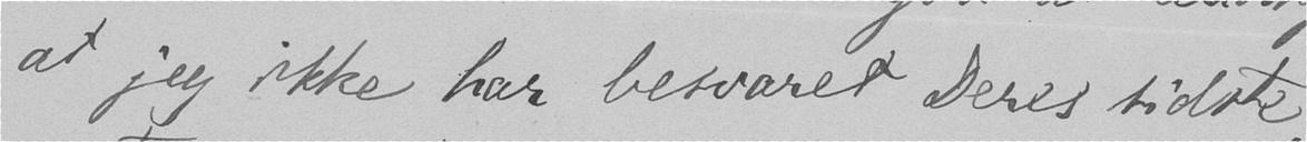at jeg ikke har besvaret Deres sidste,
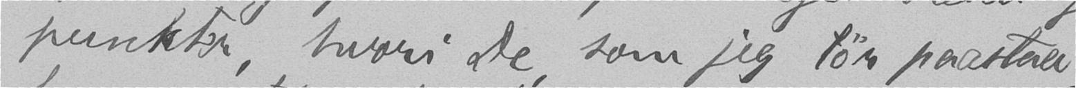punkter, hvori De, som jeg tør paastaa,
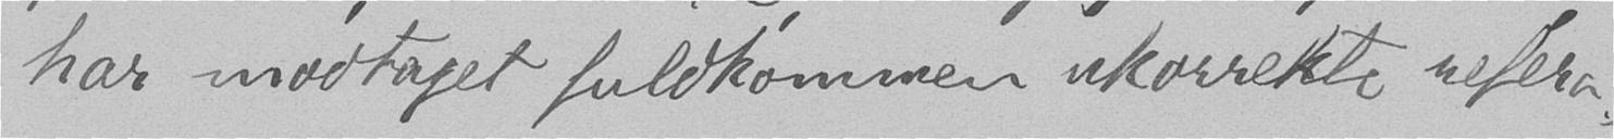har modtaget fuldkommen ukorrekte refera-
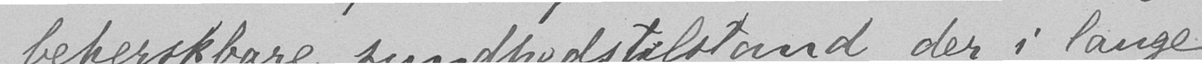beherskbare sundhedstilstand, der i lange
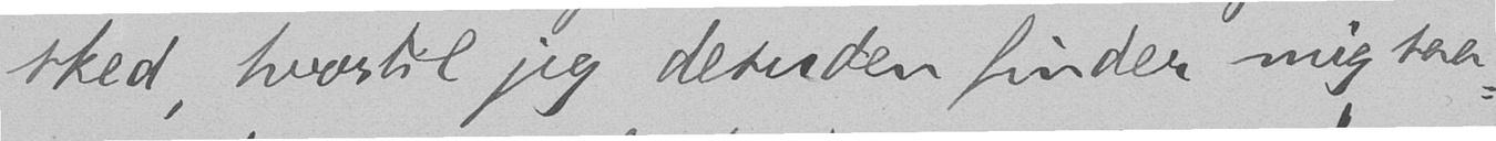sked, hvortil jeg desuden finder mig saa
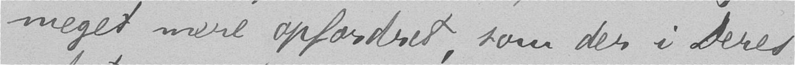meget mere opfordret, som der i Deres
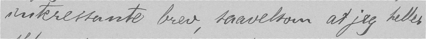interessante brev, saavelsom at jeg heller
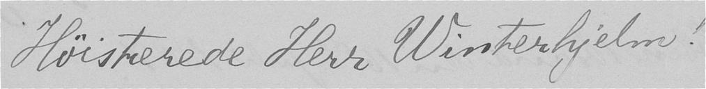Høistærede Herr Winterhjelm!
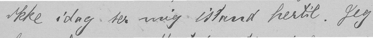ikke idag ser mig istand hertil. Jeg
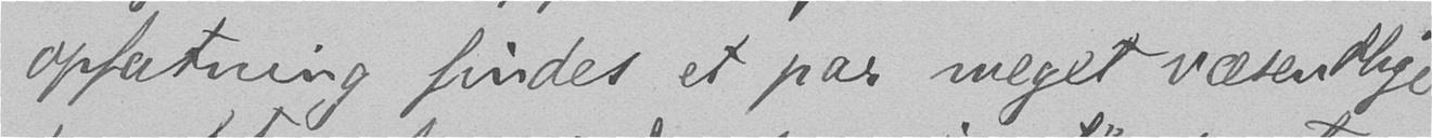opfatning findes et par meget væsentlige
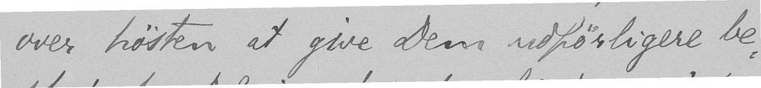over høsten at give Dem udførligere be-
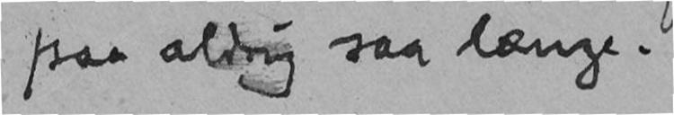paa aldrig saa længe.
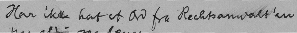Har ikke hat et Ord fra Rechtsanwalt'en
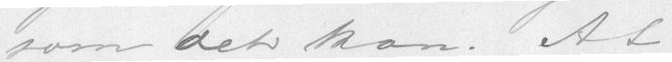som det kan. At
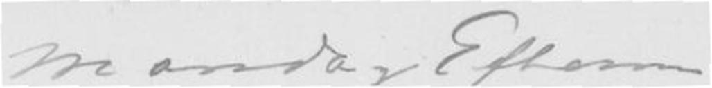Mandag Efterm.
